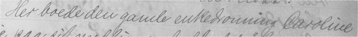Her boede den gamle enkedronning Caroline
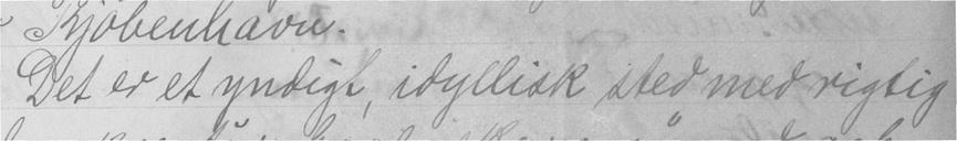Det er et yndigt, idyllisk sted med rigtig
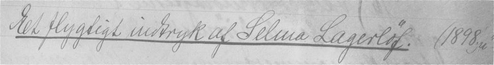Et flygtigt indtryk af Selma Lagerløf. (1898)
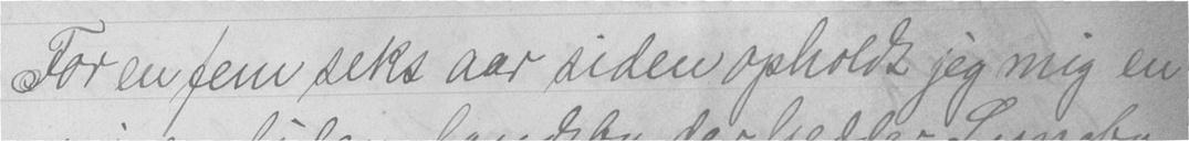For en fem seks aar siden opholdt jeg mig en
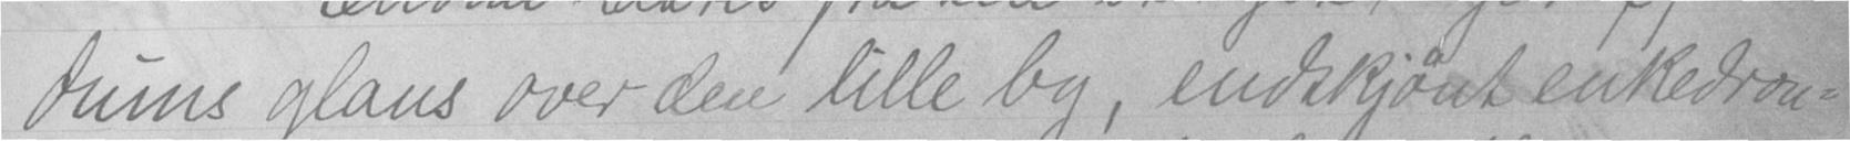dums glans over den lille by, endskjønt enkedron-
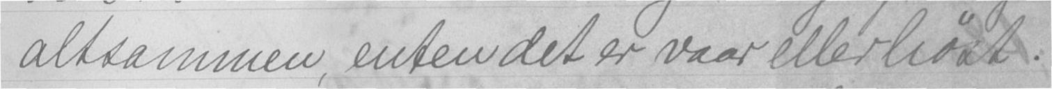altsammen, enten det er vaar eller høst.
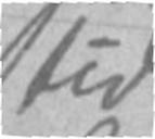tid
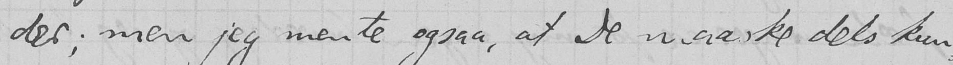der; men jeg mente ogsaa, at De maaske dels kun-
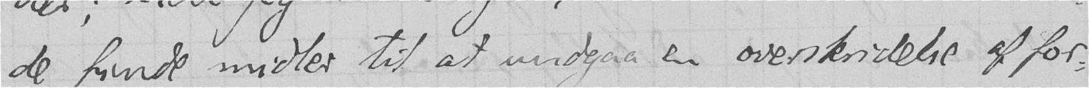de finde midler til at undgaa en overskridelse af for-
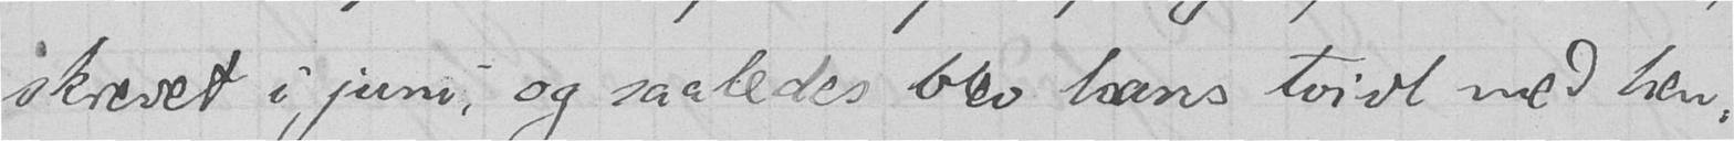skrevet i juni, og saaledes blev hans tvivl med hen-
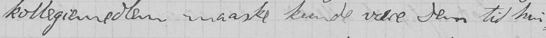kollegiemedlem maaske kunde være Dem til hin-
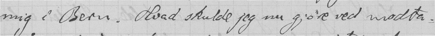mig i Bern. Hvad skulde jeg nu gjøre ved modta-
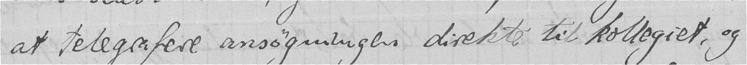at telegrafere ansøgningen direkte til kollegiet, og
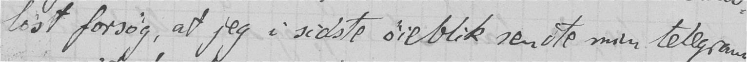løst forsøg, at jeg i sidste øieblik sendte min telegram;
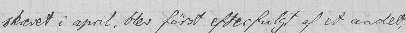skrevet i april, blev først efterfulgt af et andet,
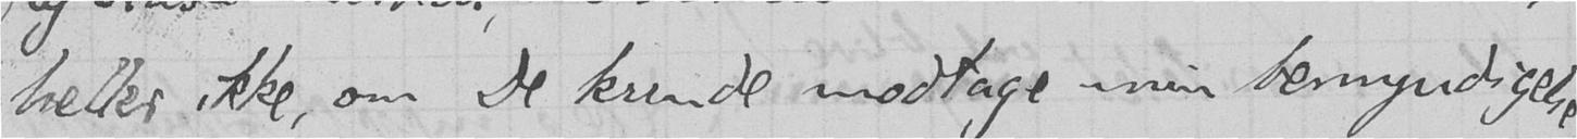heller ikke, om De kunde modtage min bemyndigelse,
